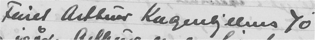Feiret Arthur Knagenhjelms 70
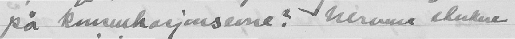på konsentrasjonsevne? Nervene sterkere
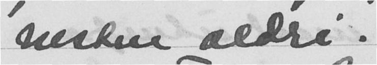nesten aldri.
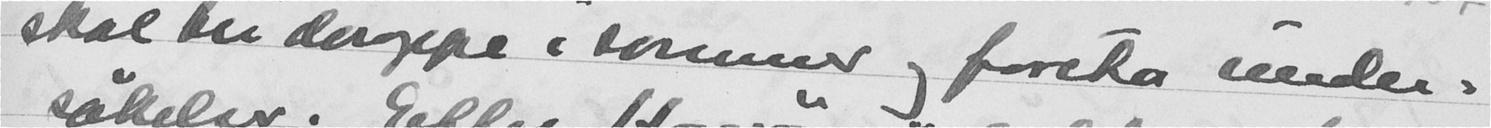skal bli deroppe i sommer og foreta under-
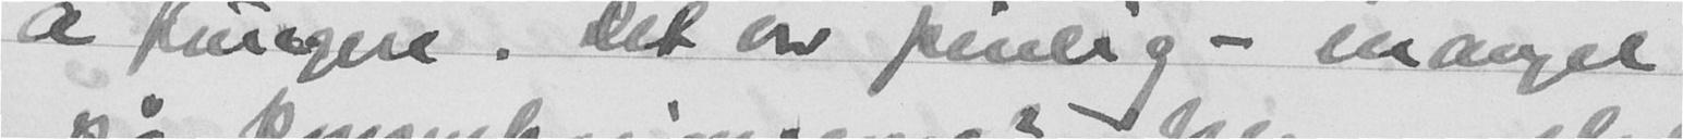å fungere. Det er pinlig - mangel
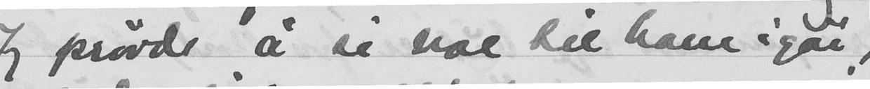Jeg prøvde å se noe til ham igår,
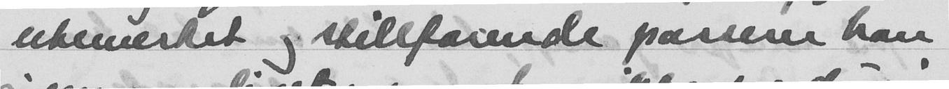ubemerket og stillfarende passerer han
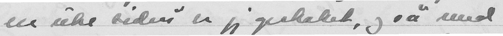en uke siden er jeg opskaket, og så med
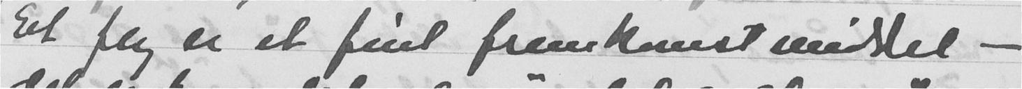Et fly er et fint fremkomstmiddel -
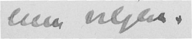enn viljen.
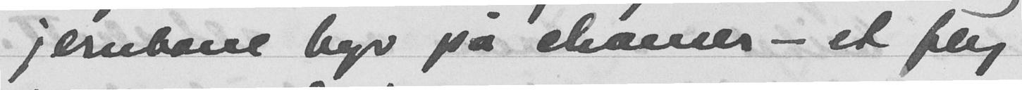jærnbane byr på charme - et fly
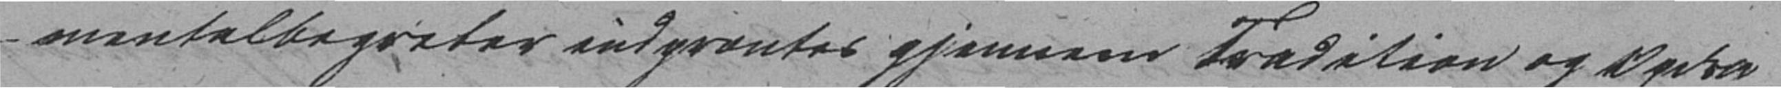mentalbegreber indprentes gjennem Tradition og Opdra-
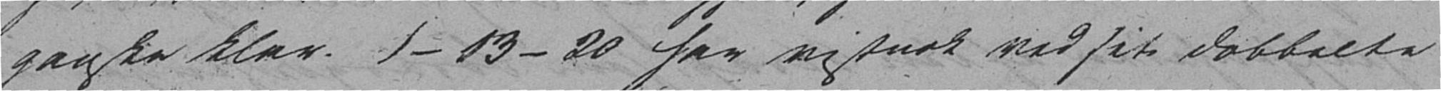ganske klar. 1-13-20 har vistnok ved sit dobbelte
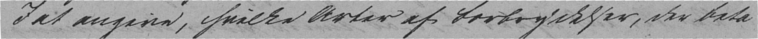I at angive, hvilke Arter af Forbrydelser, der beta-
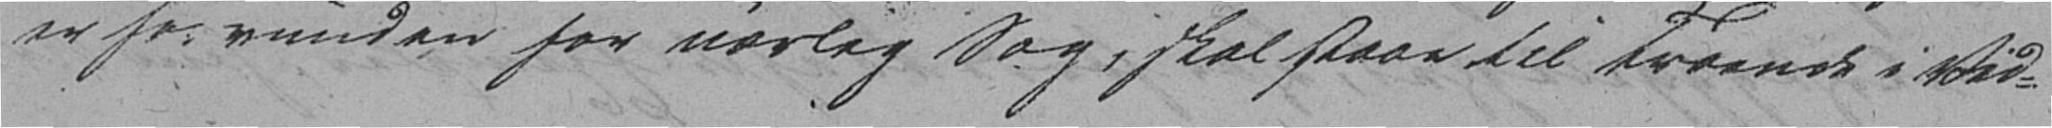er forvunden for uærlig Sag, skal staae til Troende i Vid-
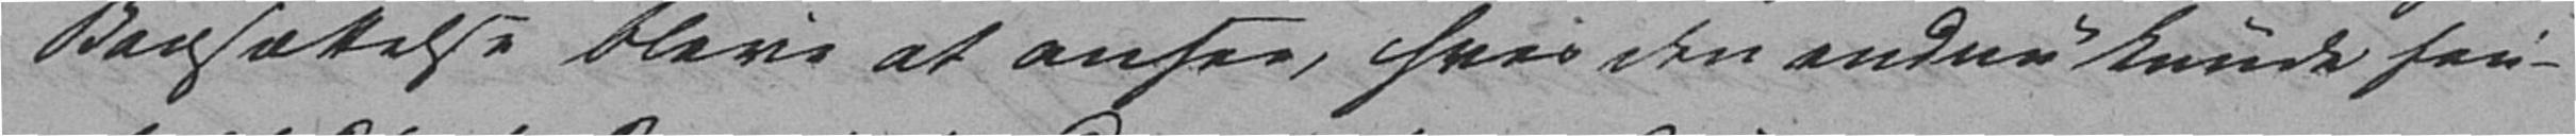Besættelse blive at ansee, hvis den endnu kunde fin-
